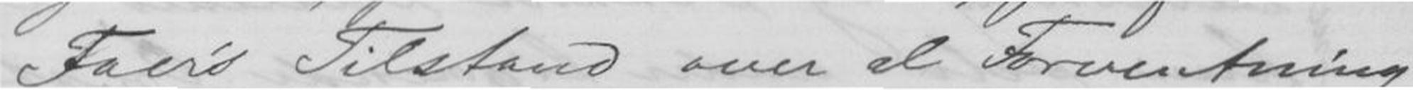Faer's Tilstand over al Forventning
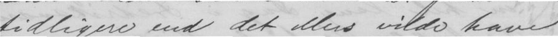tidligere end det ellers vilde have
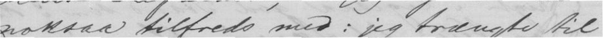noksaa tilfreds med: jeg trængte til
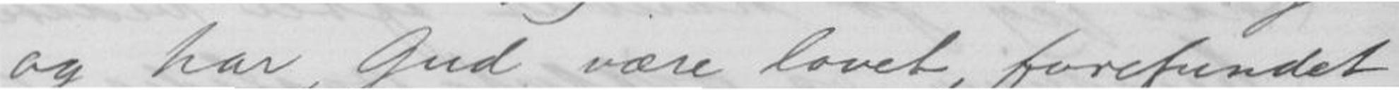og har, Gud være lovet, forefundet
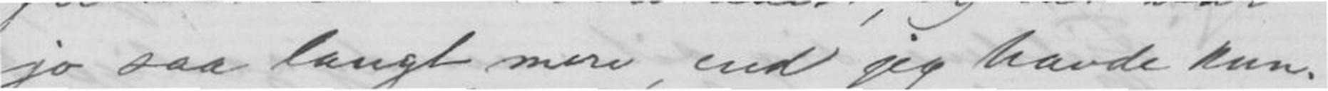jo saa langt mere, end jeg havde kun-
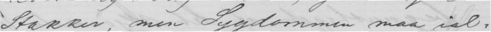Stakker, men Sygdommen maa ial-
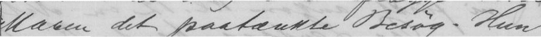Maren det paatænkte Besøg. Hun
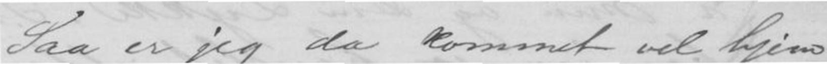Saa er jeg da kommet vel hjem
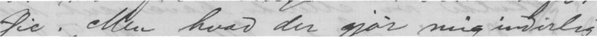Øie. Men hvad der gjør mig inderlig
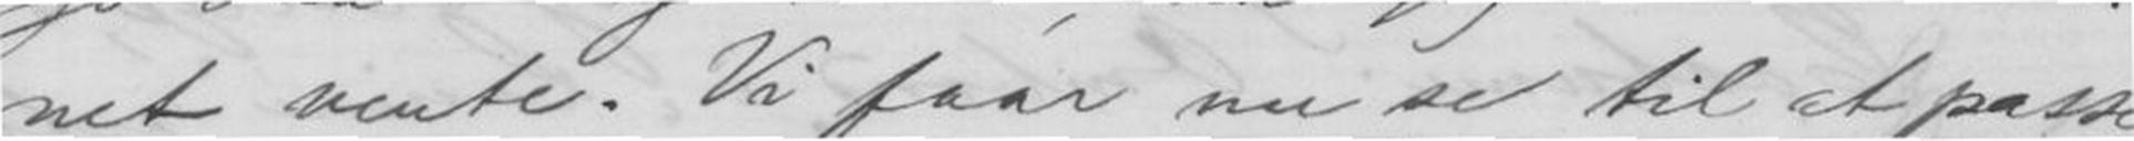net vente. Vi faar nu se til at passe
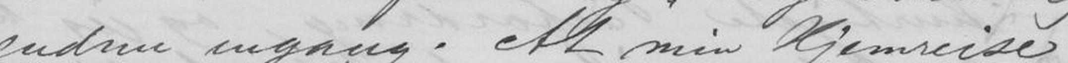endnu engang. At min Hjemreise
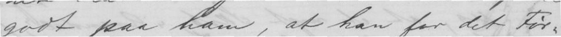godt paa ham, at han for det Før-
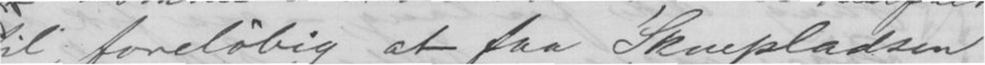til, foreløbig at faa Skuepladsen
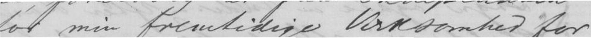for min fremtidige Virksomhed for
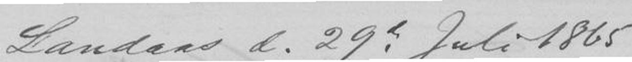Landaas d. 29de Juli 1865
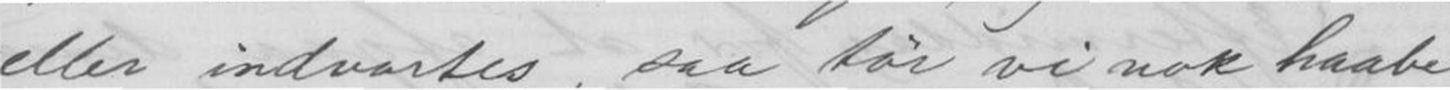eller indvortes, saa tør vi nok haabe
